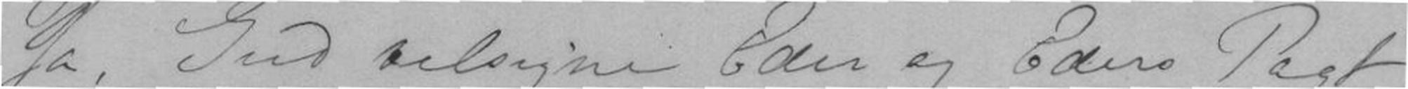Ja, Gud velsigne Eder og Eders Pagt
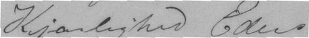Kjærlighed Eders
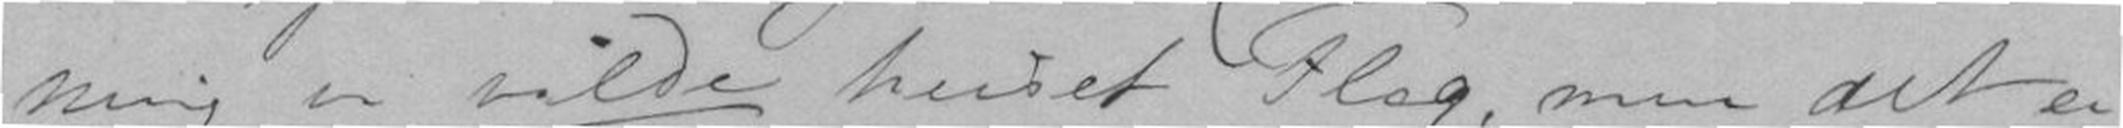ning vi vilde heiset Flag, men det er
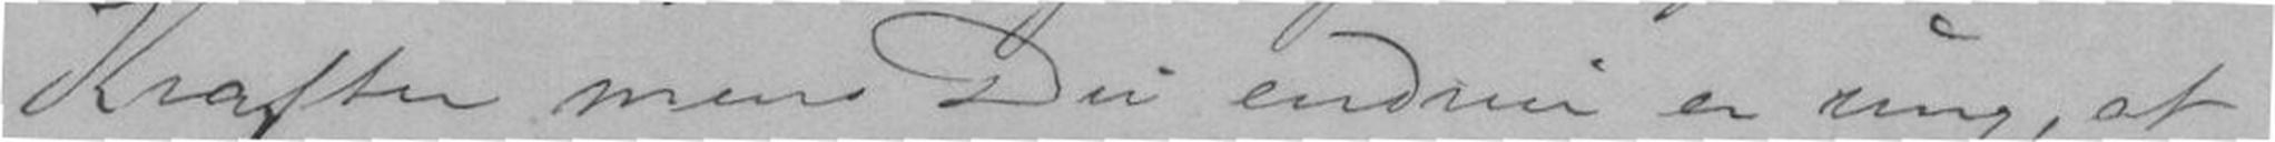Kræfter mens Du endnu er ung, at
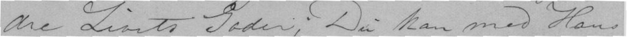dre Livets Goder; Du kan med Hans
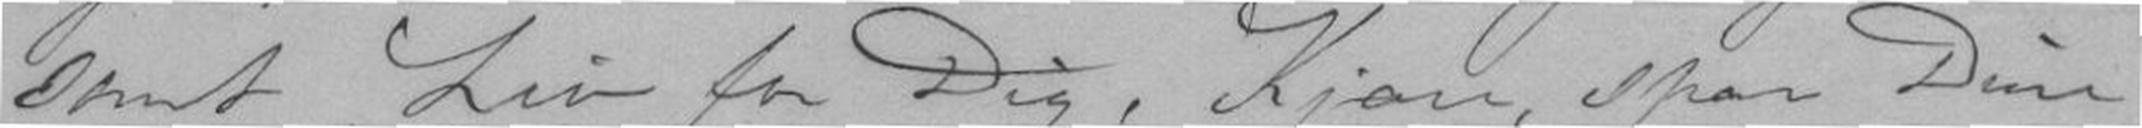somt Liv for Dig; Kjære spar Dine
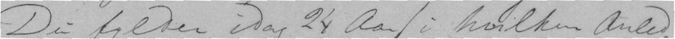Du fylder idag 24 Aar (i hvilken Anled-
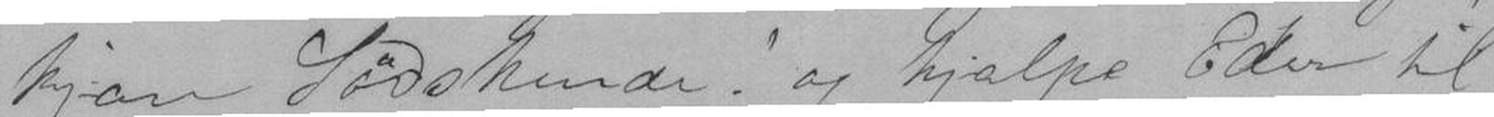kjære Sødskende! og hjælpe Eder til
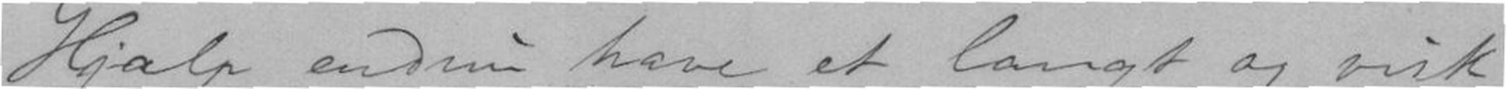Hjælp endnu have et langt og virk-
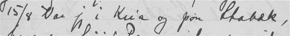15/8 Var jeg i Kria og paa Stabæk,
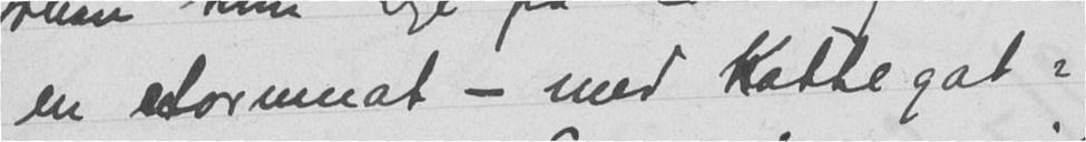en stormnat - med Kattegat-
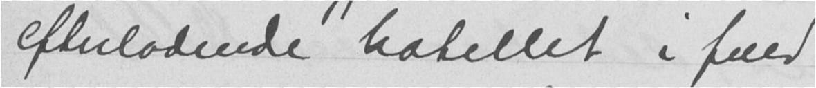efterladende hotellet i fuld
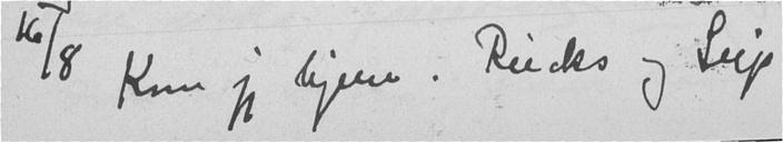16/8 Kom jeg hjem. Riecks og Seip
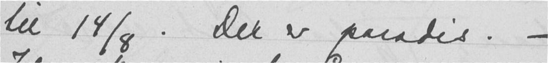til 14/8. Der er paradis. -
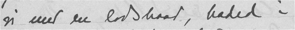vi med en lodsbaad, baded i
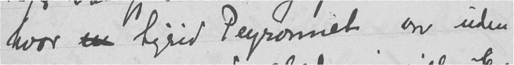hvor en Sigrid Peyronnet var uden
